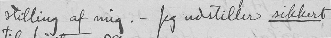stilling af mig. - Jeg udstiller sikkert
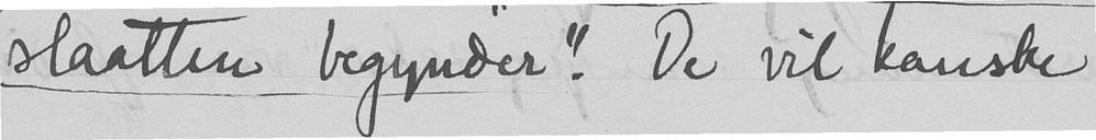slaatten begynder". De vil kanske
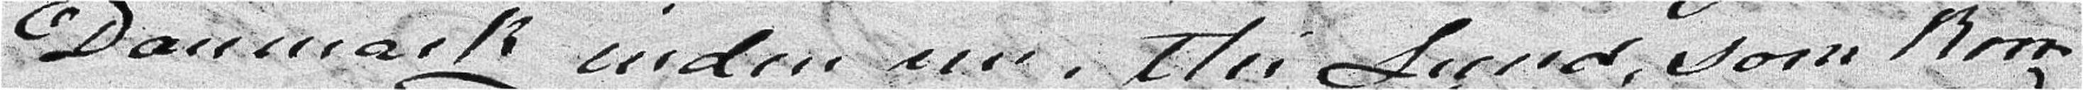Danmark inden nu, thi Lund, som kom
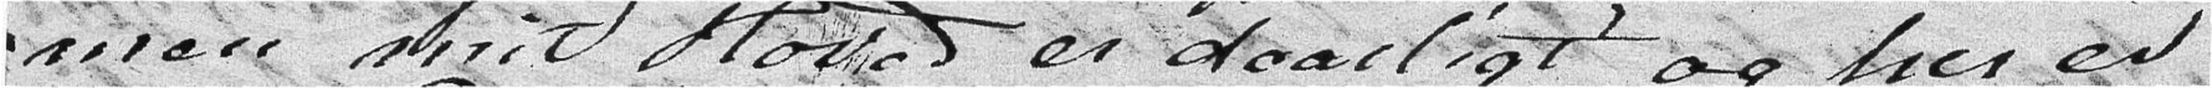men mit Hoved er daarligt og her er
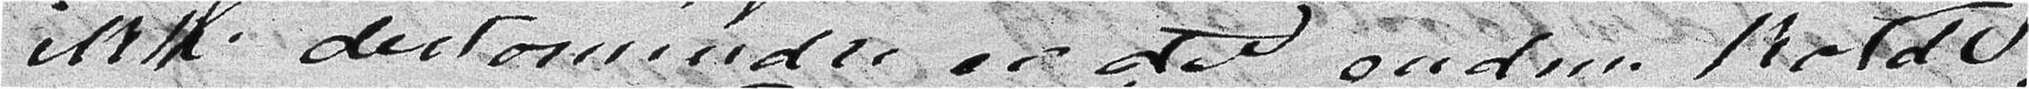ikke destomindre er det endnu koldt,
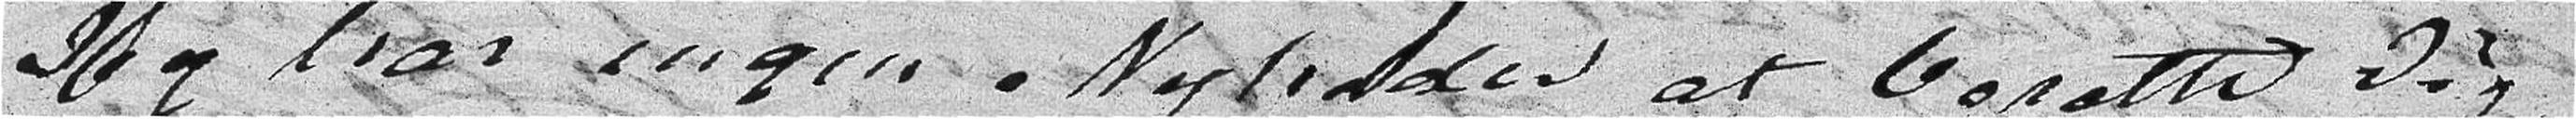Jeg har ingen Nyheder at berette Dig,
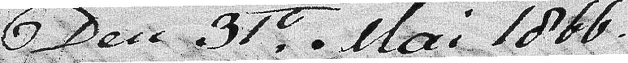Den 31t Mai 1866.
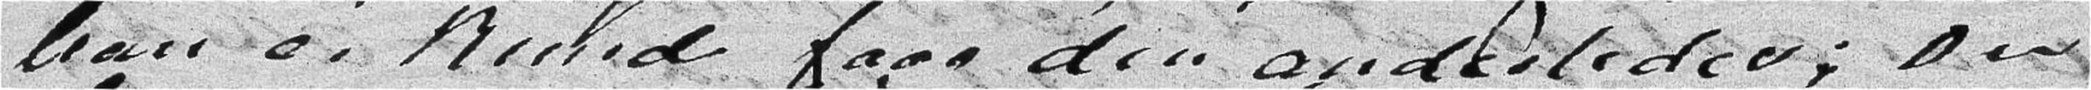han ei kunde faae den anderledes; Du
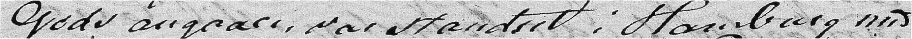Gods angaaer, var standset i Hamburg med
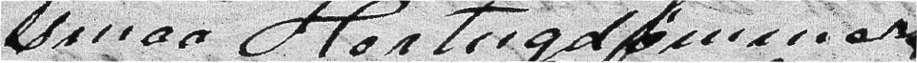smaa Hertugdømmer
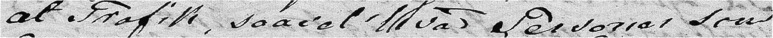al Trafik, saavel hvad Personer, som
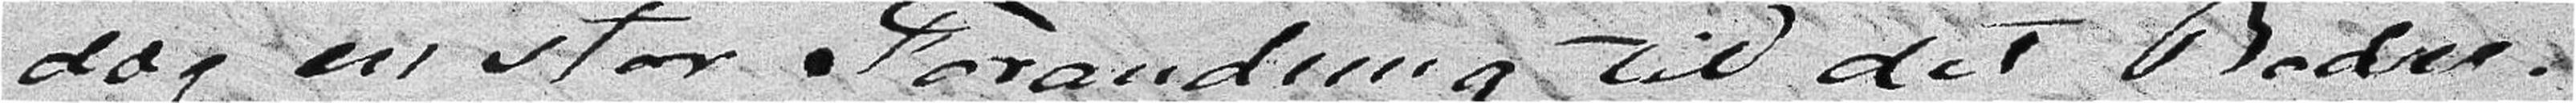dog en stor Forandring til det Bedre.
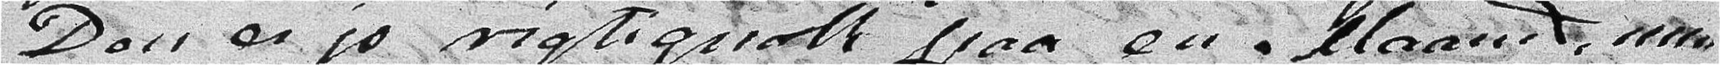Den er jo rigtignok paa en Maaneed, men
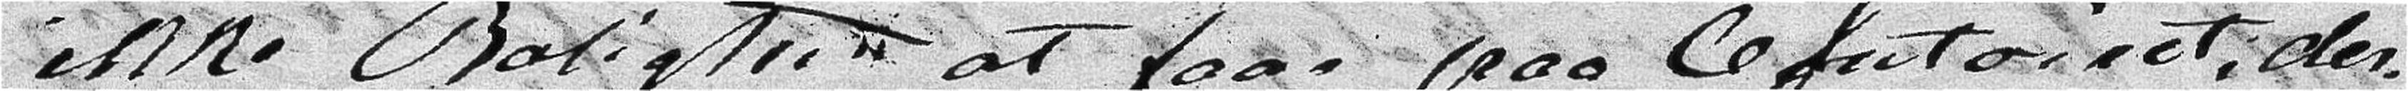ikke Rolighed at faae paa Contoiret, der-
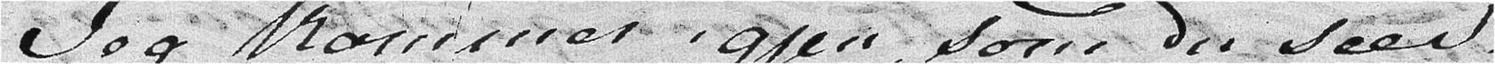Jeg kommer igjen som Du seer,
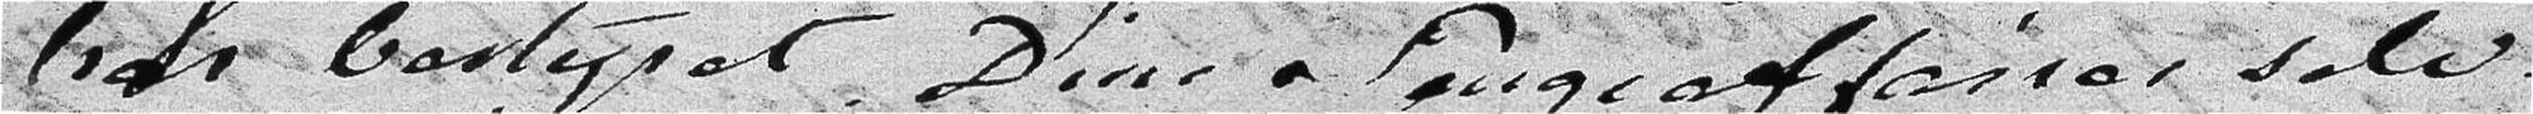har bestyret Dine Pengeaffairer selv.
